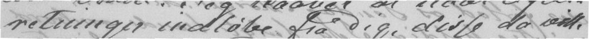retninger indløbe fra Dig, disse da ville
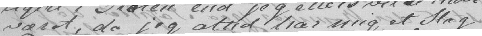været, da jeg altid har mig et Slag
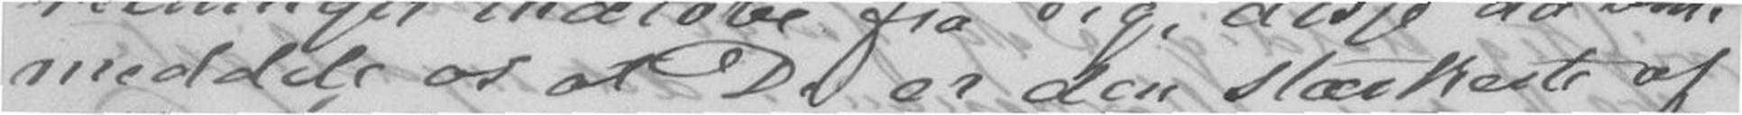meddele os at Du er den stærkeste af
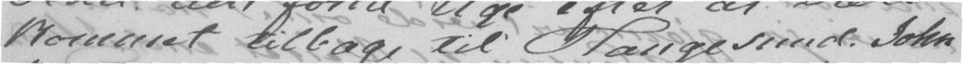kommet tilbage til Haugesund. John
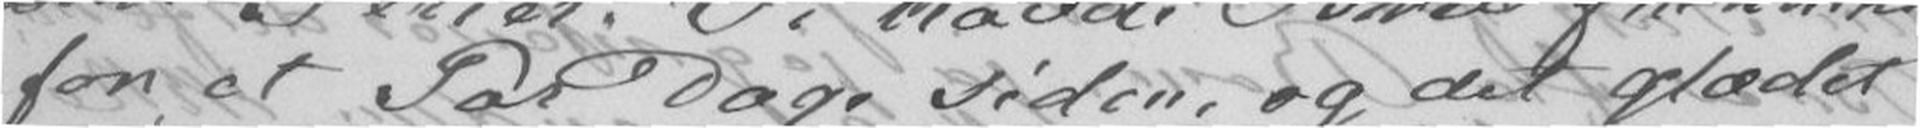for et Par Dage siden, og det glædet
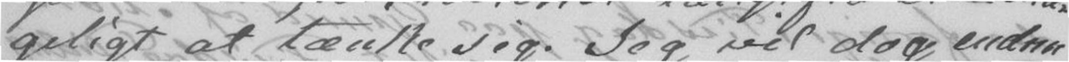geligt at tænke sig. Jeg vil dog endnu
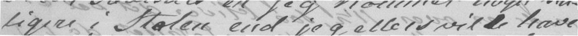ligere i Stolen end jeg ellers vilde have
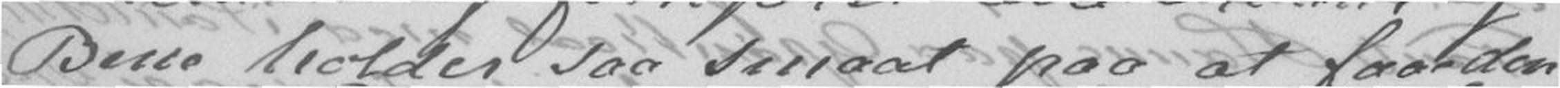Bene holder saa smaat paa at faae den
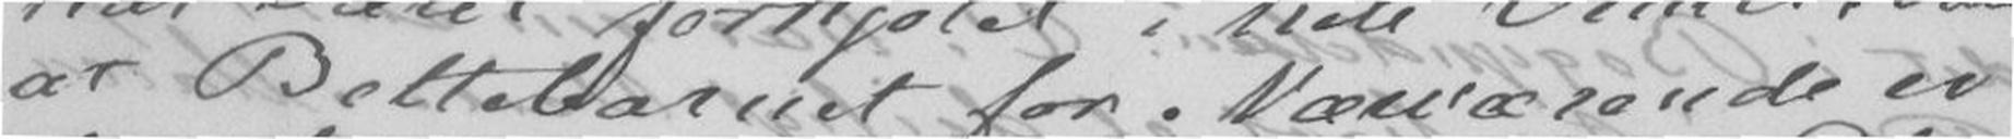at Bettebarnet for Nærværende er
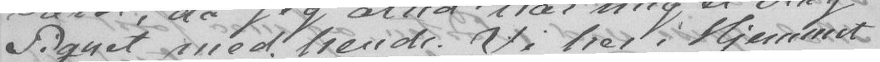Piqnet med hende. Vi her i Hjemmet
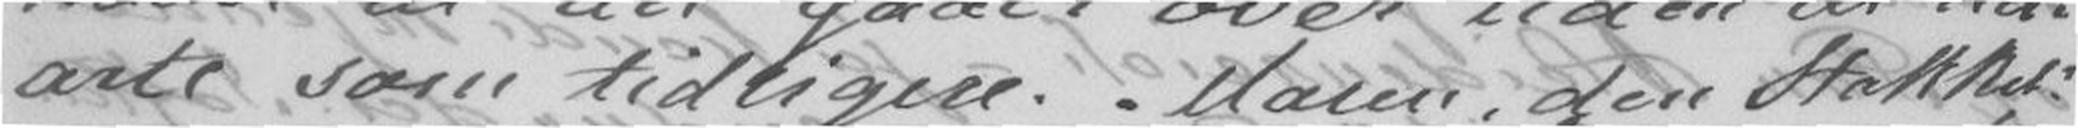arte som tidligere. Maren den stakkels
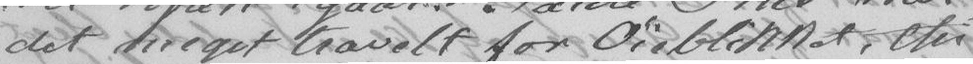det meget travelt for Øieblikket, thi
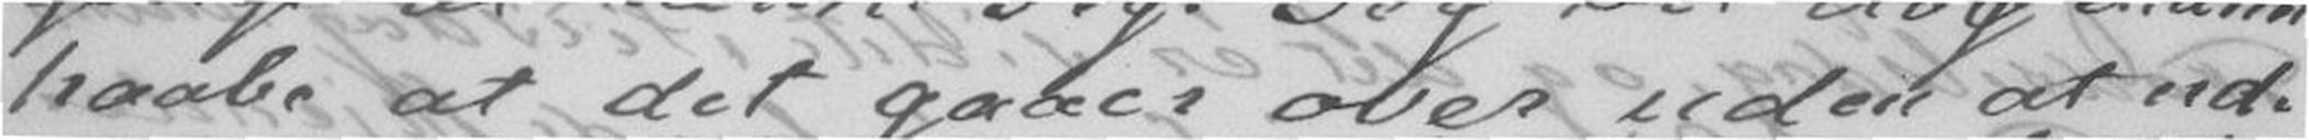haabe at det gaaer over uden at ud-
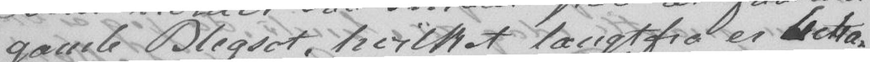gamle Blegsot, hvilket langtfra er beha-
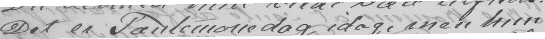Det er Tantemonedag idag, men hun
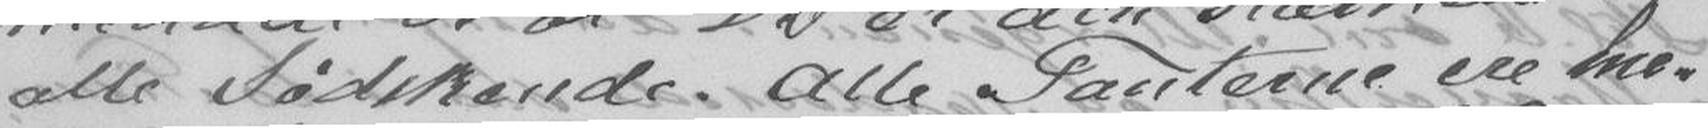alle Sødskende. Alle Tanterne ere me-
